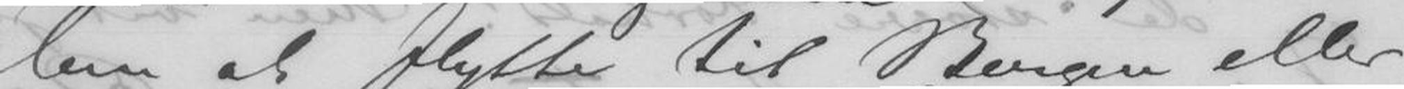lem at flytte til Bergen eller
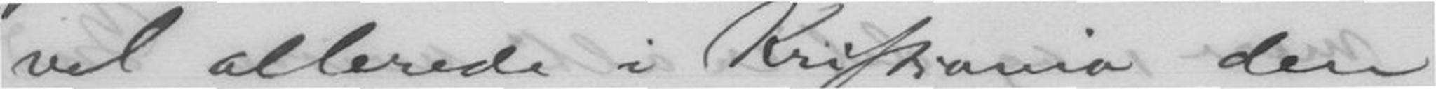vel allerede i Kristiania den
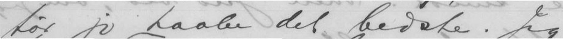tør jo haabe det bedste. Jeg
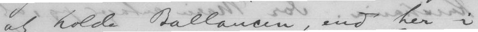at holde Ballancen, end her i
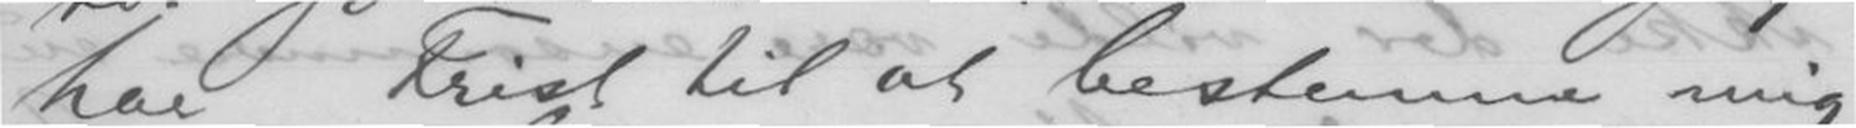har Frist til at bestemme mig
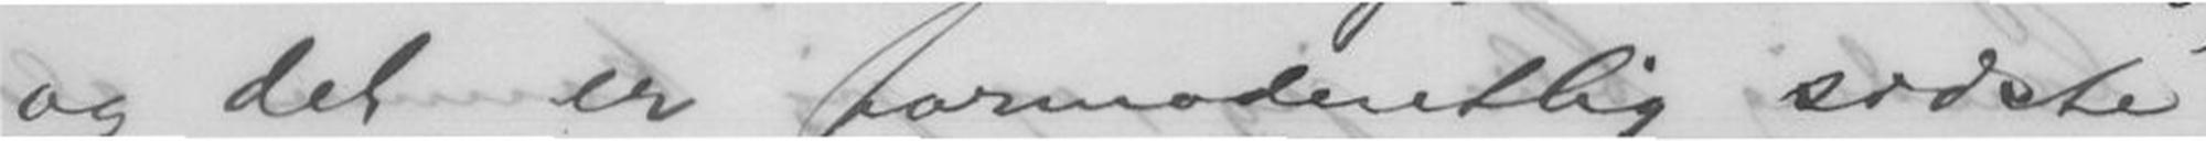og det er formodentlig sidste
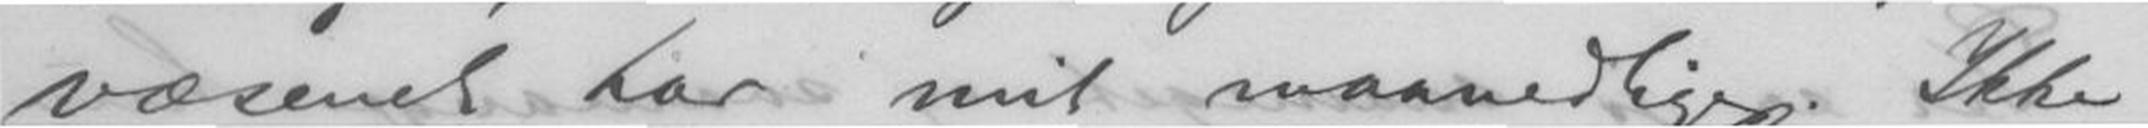væsenet har mit maanedlige. Ikke
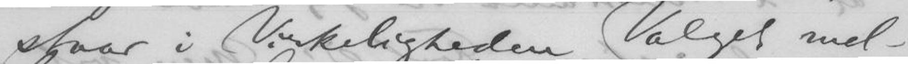staar i Virkeligheden Valget mel-
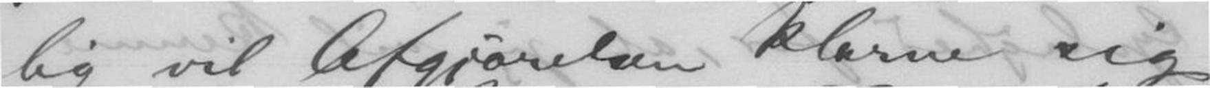lig vil Afgjørelsen klarne sig
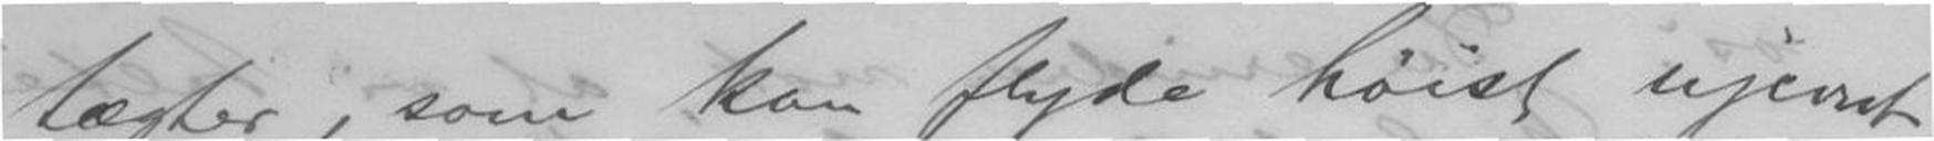tægter, som kan flyde høist ujevnt,
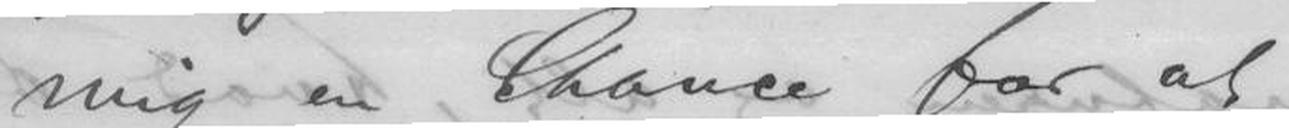mig en Chance for at
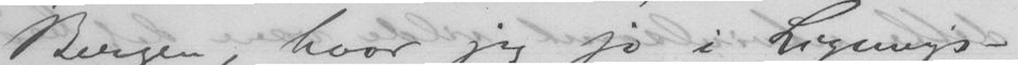Bergen, hvor jeg jo i Lignings-
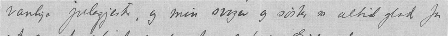vanlige julegjester, og min svoger og søster er altid glad for
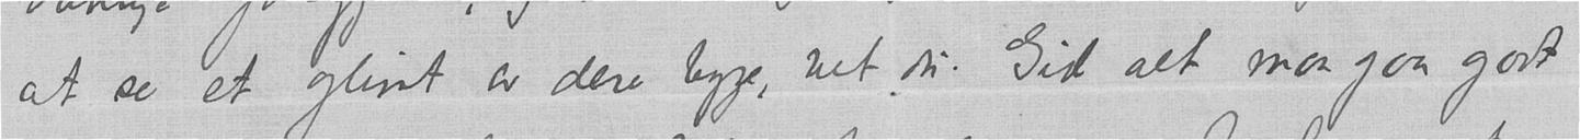at se et glimt av dere begge, vet du. Gid alt maa gaa godt
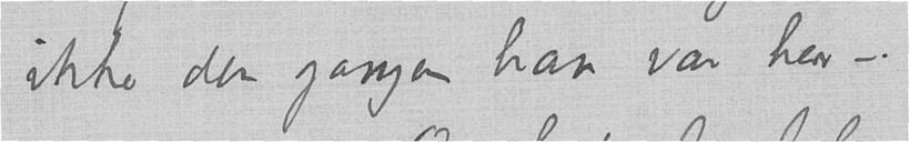ikke den gangen han var her –.
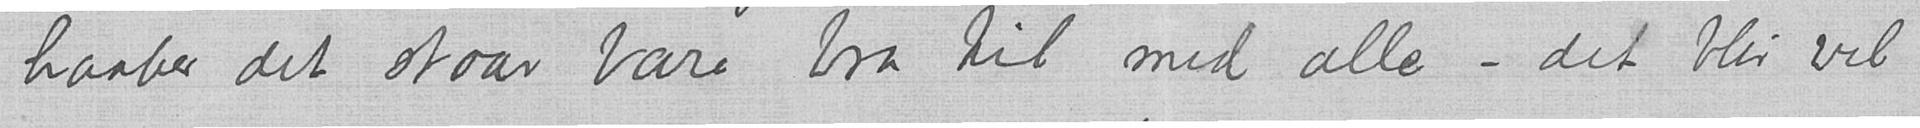haaber det staar bare bra til med alle – det blir vel
